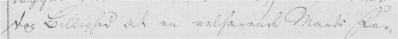dog Billighed at en velhavende Mands For-
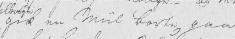gik en Miil borte paa
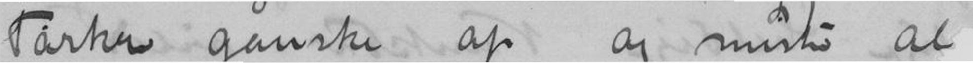Tørker ganske op og mister al
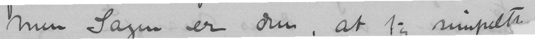Men Sagen er den, at jeg simpelt
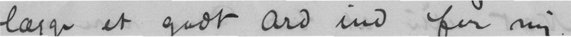lægge et godt Ord ind for mig.
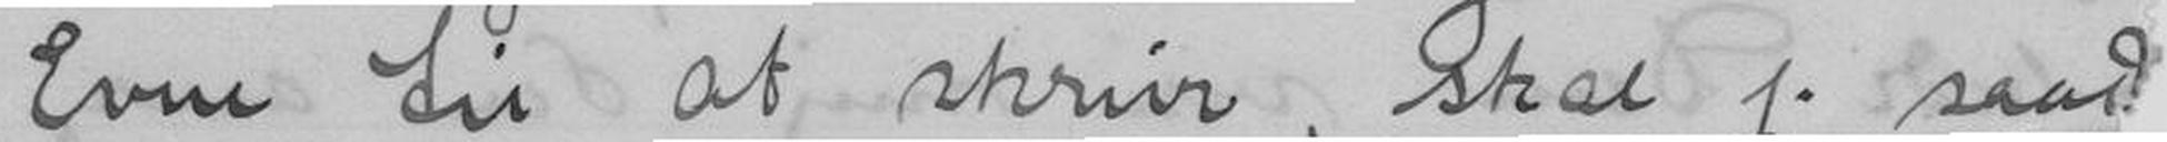Evne til at skrive, skal jeg saad
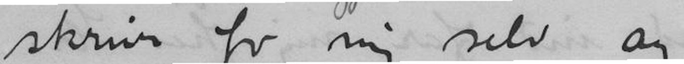skriver for mig selv og
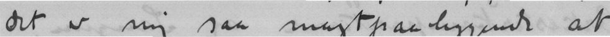det er mig saa magtpaaliggende at
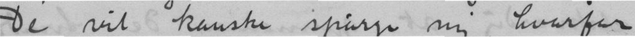De vil kanske spørge mig, hvorfor
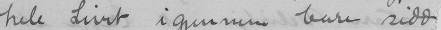hele Livet igjennem bare sidd
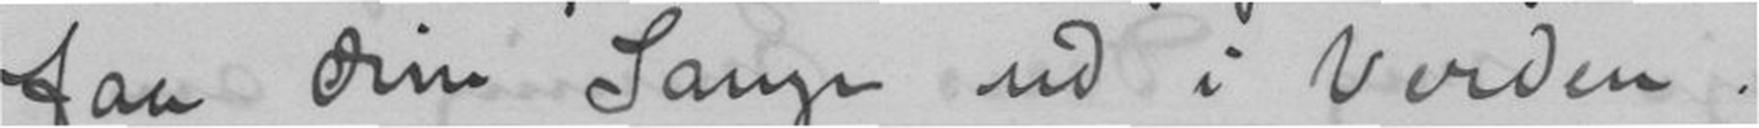faa dine Sange ud i Verden.
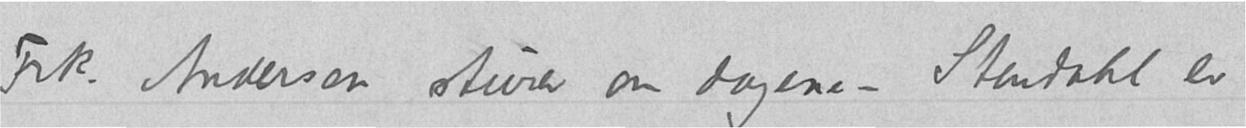Frk. Andersen sturer om dagene – Stendahl er
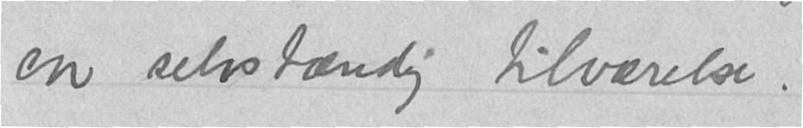en selvstændig tilværelse.
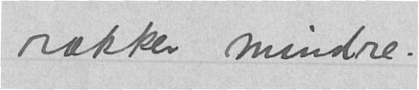rækker mindre.
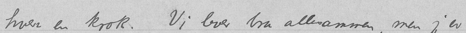hver en krok. Vi lever bra allesammen, men jeg er
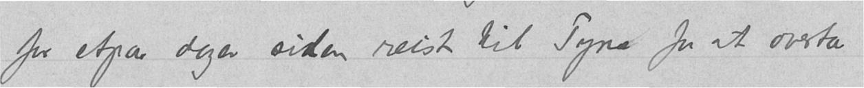for etpar dager siden reist til Tyne for at overta
